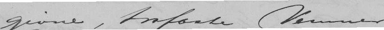givne, trofaste Venner
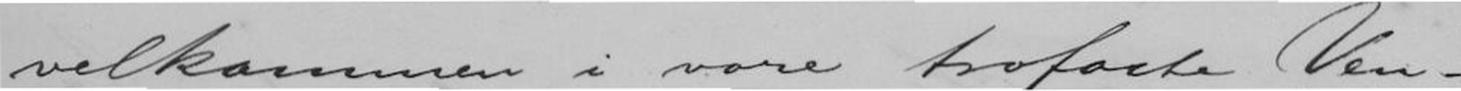velkommen i vore trofaste Ven-
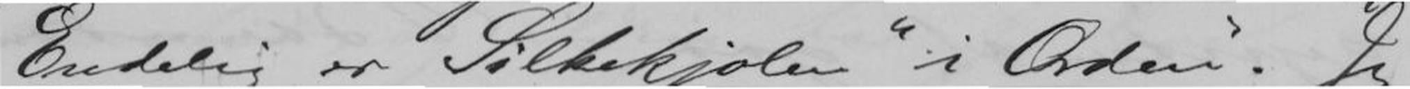Endelig er Silkekjolen i Orden. Jeg
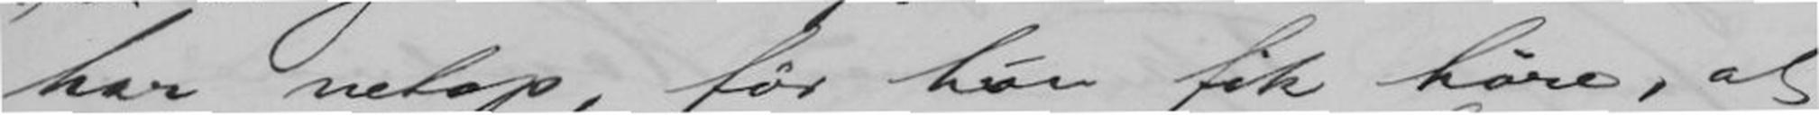har netop, før hun fik høre, at
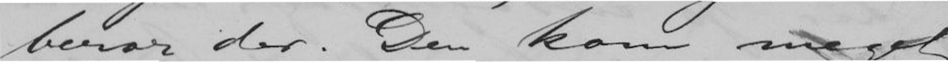beror der. Den kom meget
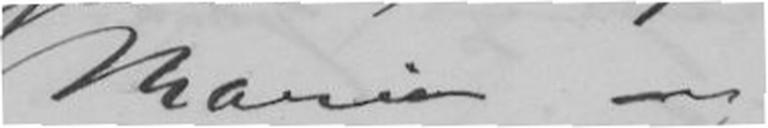Marie og
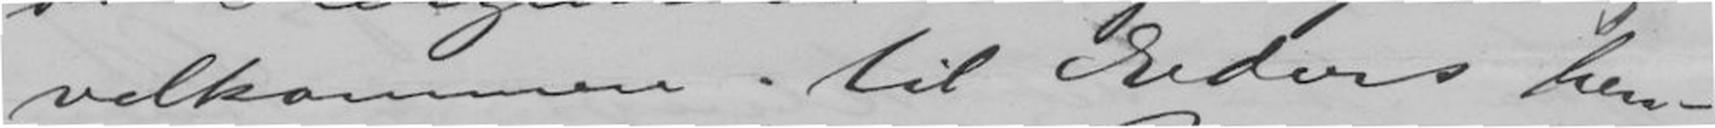velkommen til Eders hen-
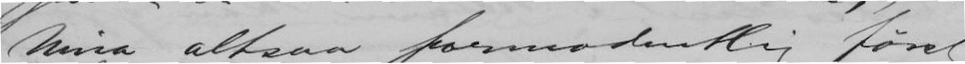Nina altsaa formodentlig først
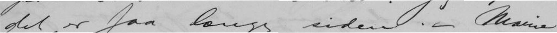det er saa længe siden. - Marie
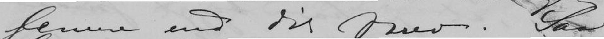senere end dit Brev. Saa
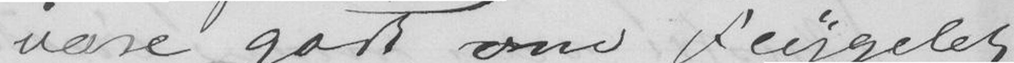være godt om Flygelet
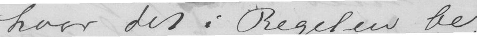hvor det i Regelen be
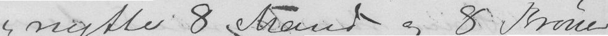nytte 8 Mand og 8 Kroner
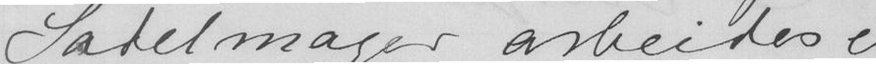Sadelmager arbeides et
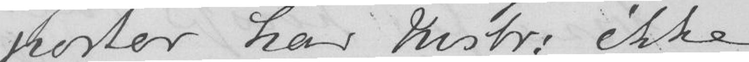porter har Instr: ikke
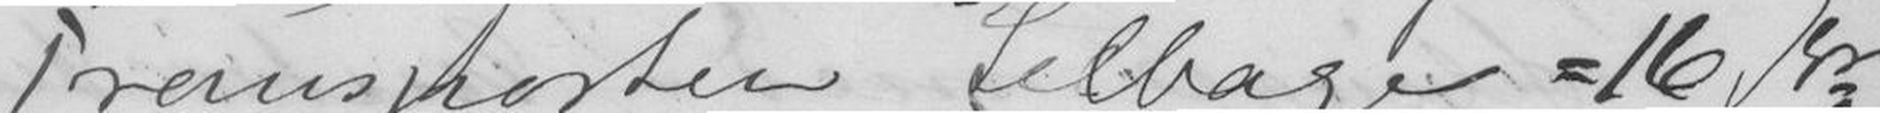Transporten tilbage = 16 Kr
